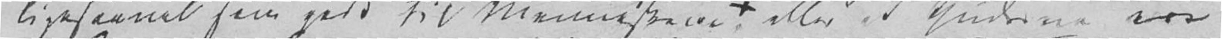ligesaavel som godt til Menneskene eller at Guderne ere
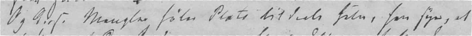Og disse Mangler føler Plato til Deels selv, han siger, at
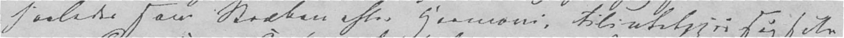saaledes som Stræben efter Harmoni, tilintetgjør sig selv
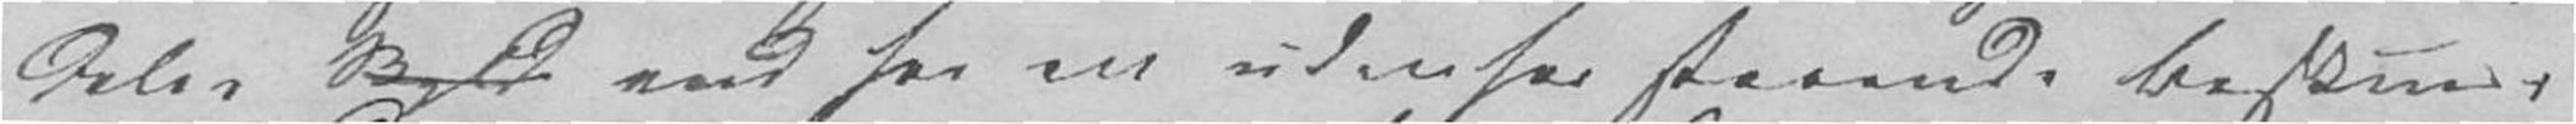Deles Skyld end for en udenfor staaende Beskuers.
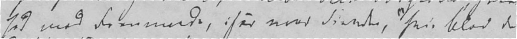hed med Fremmede, især mod Fiender, "hvis Blod de
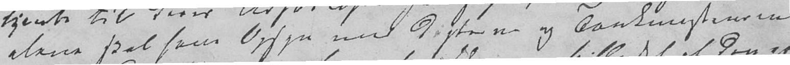alene skal have Opsyn med Digterne og Tankesystemerne
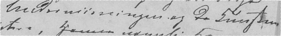Underviisningen og de Kunsten.
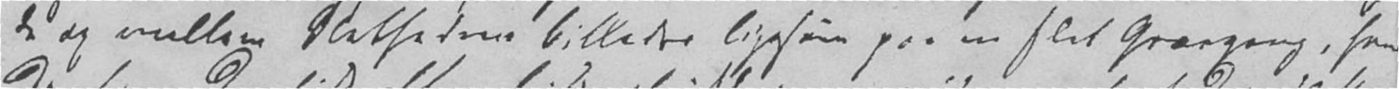de op mellem Slethedens Billeder ligesom paa en slet Græsgang, for
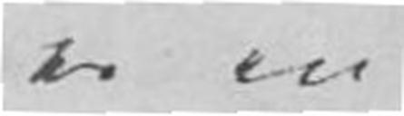er en
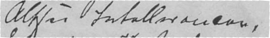Altsaa Intollerancen.
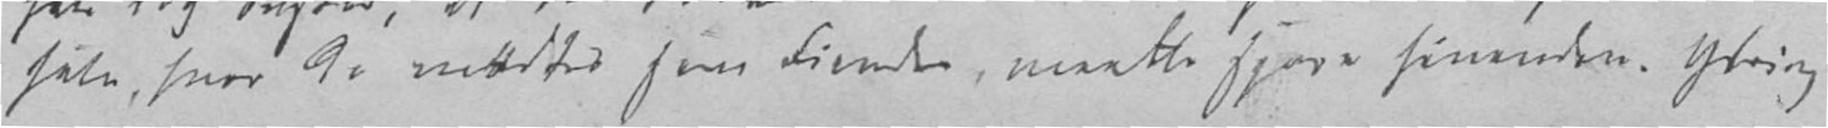selv, hvor de møttes som Fiender, maatte spare hinanden. Ytring
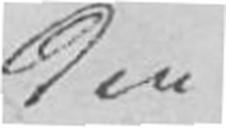den
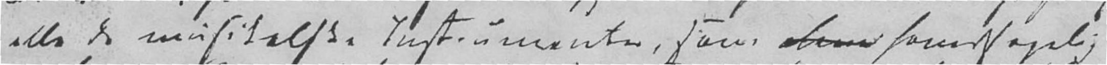alle de musikalske Instrumenter, som alene hovedsagelig
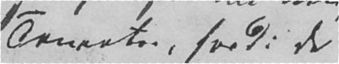Comenter, fordi de
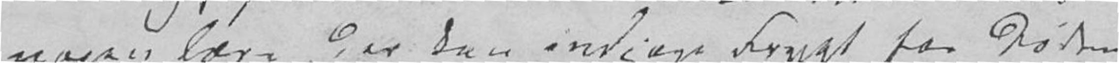nogen Lære, der kan indjage Frygt for Døden
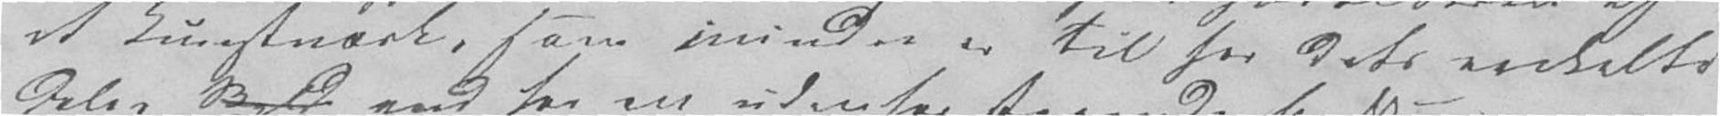et Kunstværk, som mindre er til for dets enkelte
